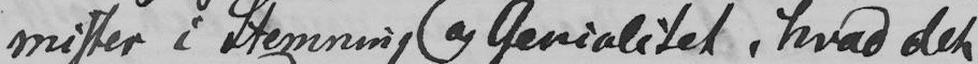mister i Stemning og Genialitet, hvad det
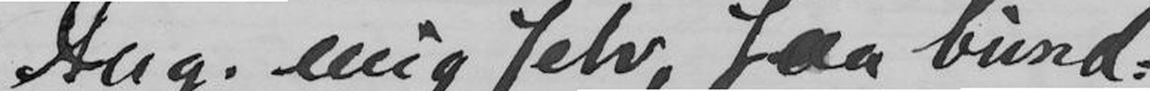Ang. mig selv, saa bund-
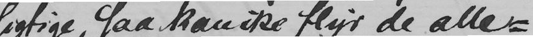sigtige, saa kanske flyr de alle-
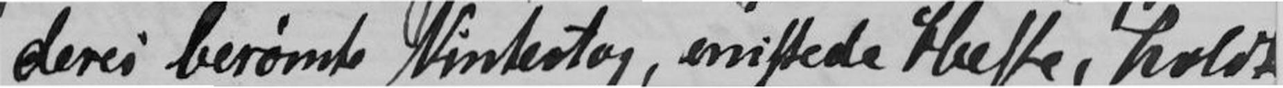deres berømte Vintertog, mistede Helse, holdt
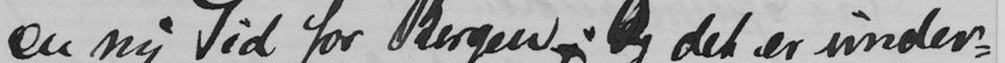en ny Tid for Bergen. Og det er under-
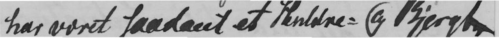har været saadant et Huldre= og Bjerg-
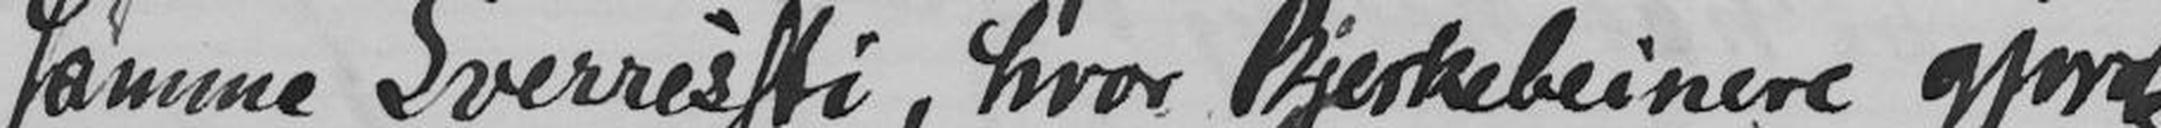samme Sverressti, hvor Bjerkebeinere gjorde
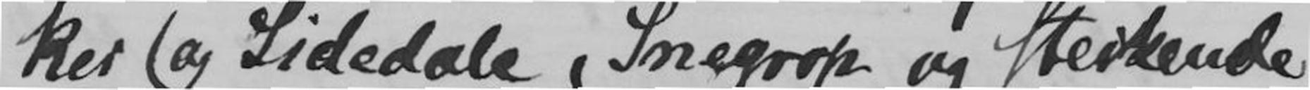ker og Sidedale, Snegrop og steikende
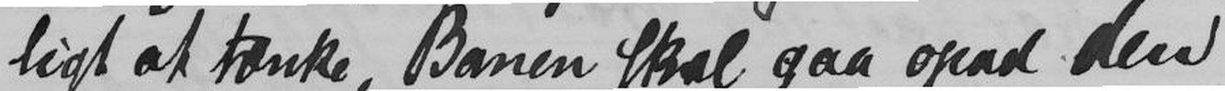ligt at tænke, Banen skal gaa opad den
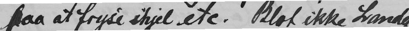paa at fryse ihjel etc. Blot ikke Lande
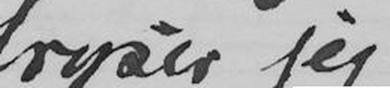fryser jeg
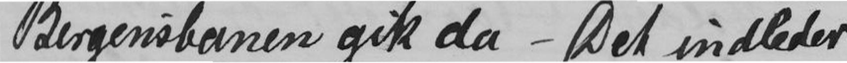Bergensbanen gik da - Det indleder
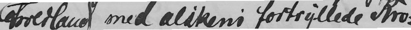Troldland med alskens fortryllede Kro-
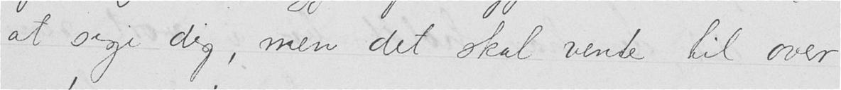at sige dig, men det skal vente til over
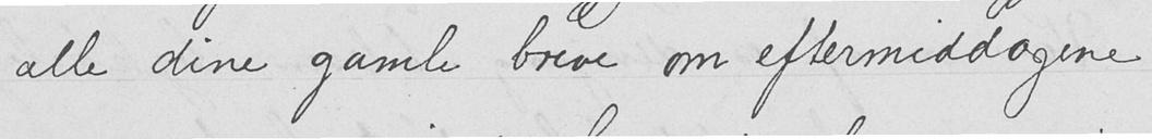alle dine gamle breve om eftermiddagene
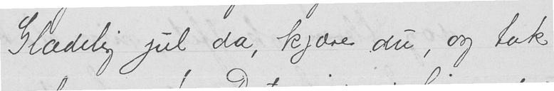Glædelig jul da, kjære du, og tak
